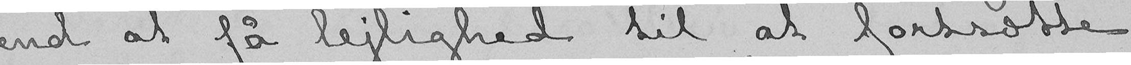end at få lejlighed til at fortsætte
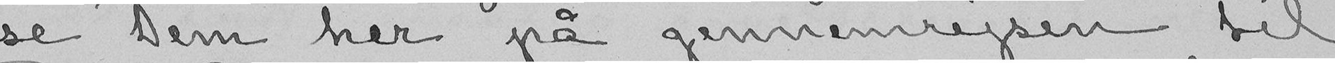se Dem her på gennemrejsen til
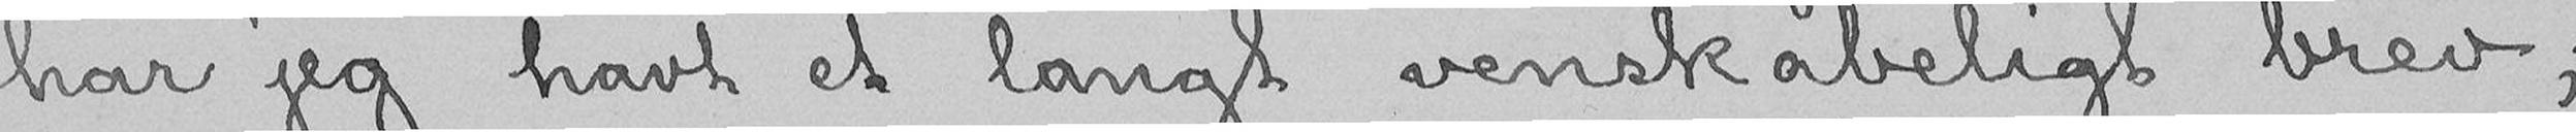har jeg havt et langt venskabeligt brev;
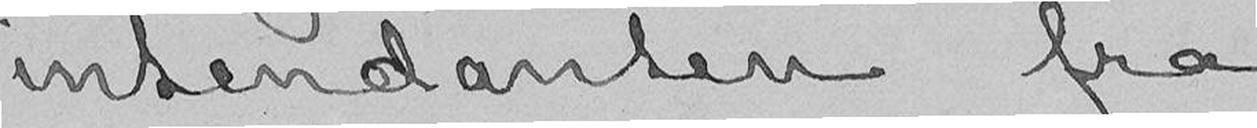intendanten fra
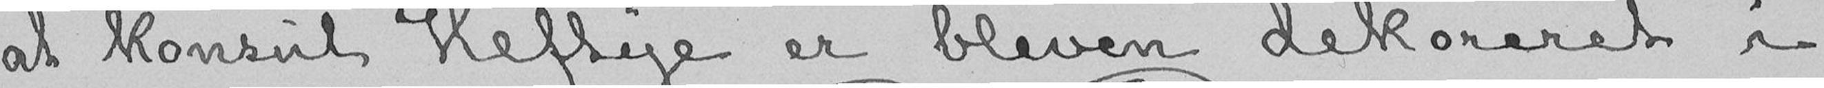at konsul Heftye er bleven dekoreret i
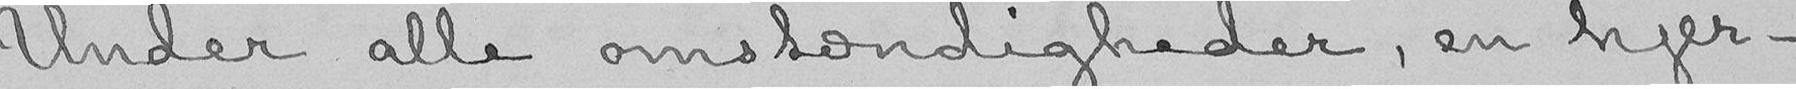Under alle omstændigheder, en hjer-
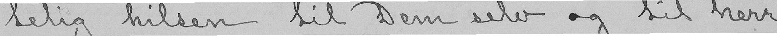telig hilsen til Dem selv og til herr
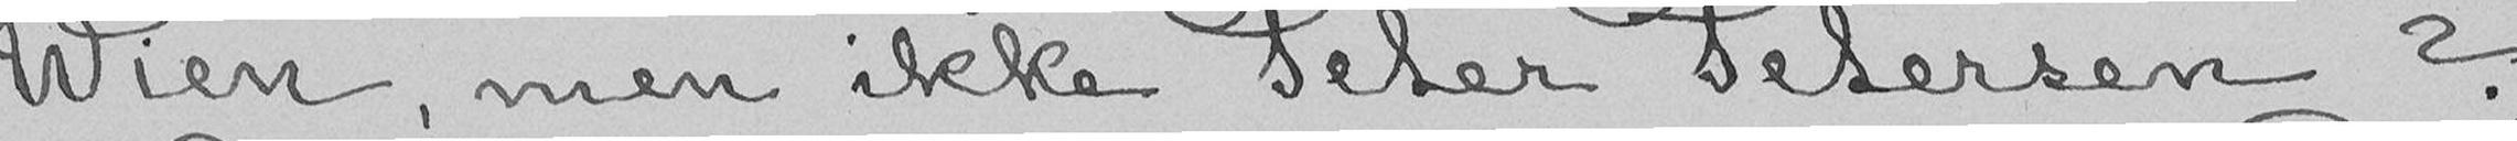Wien, men ikke Peter Petersen?
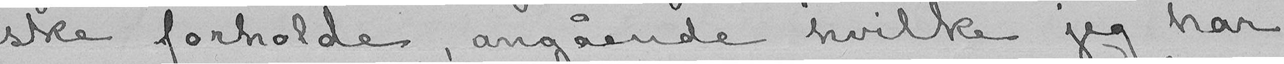ske forholde, angående hvilke jeg har
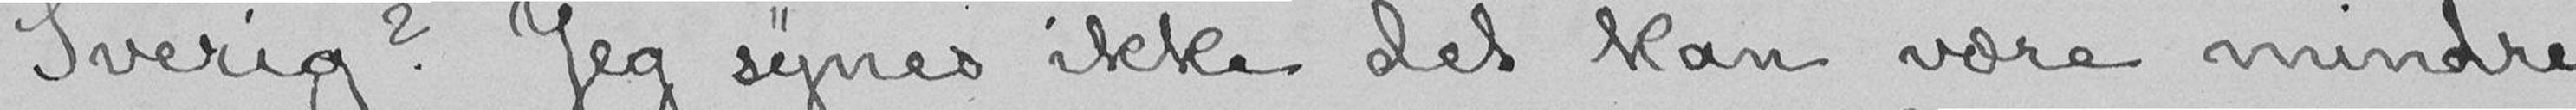Sverig? Jeg synes ikke det kan være mindre
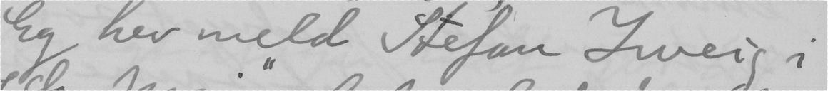Eg hev meld Stefan Zweig i
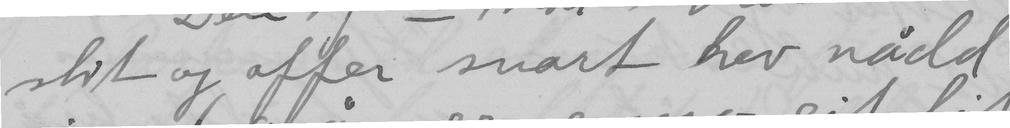slit og offer snart hev nådd
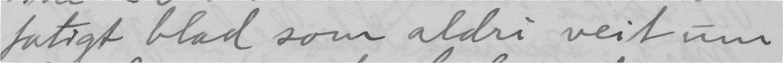fatigt blad som aldri veit um
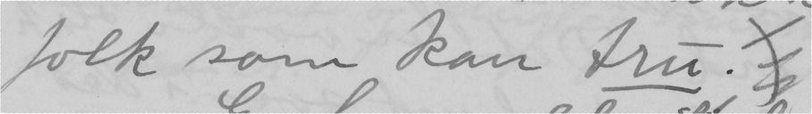folk som kan tru.
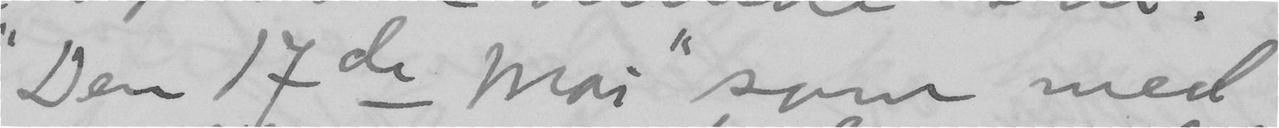"Den 17de mai" som med
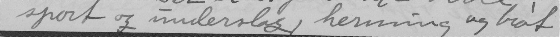sport og underslag, hemming og brót
